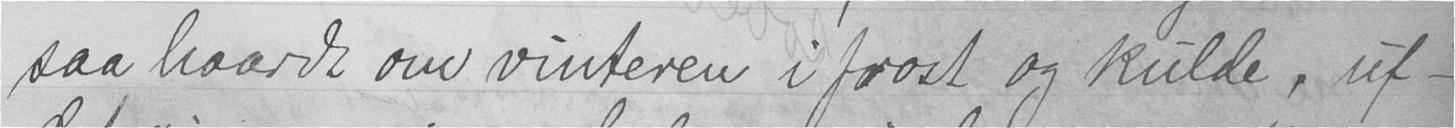saa haardt om vinteren i frost og kulde, uf -
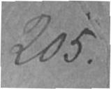205.
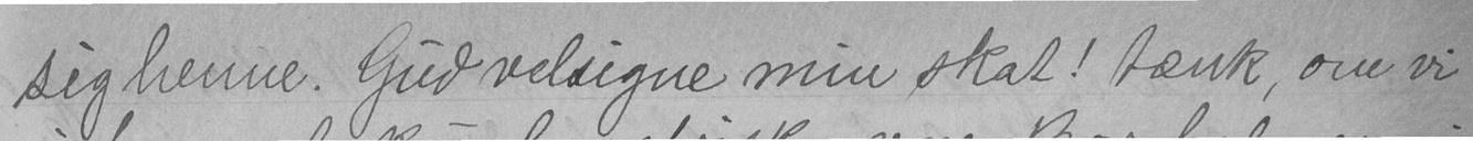sig henne. Gud velsigne min skat! tænk, om vi
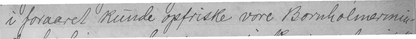i foraaret kunde opfriske vore Bornholmermin-
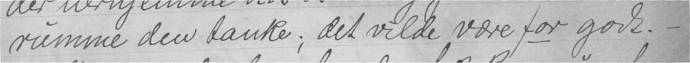rumme den tanke; det vilde være for godt. -
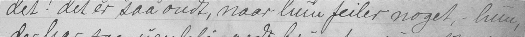det! det er saa ondt, naar hun feiler noget, - hun
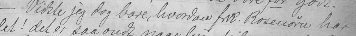- Vidste jeg dog bare, hvordan frk. Rosenørn har
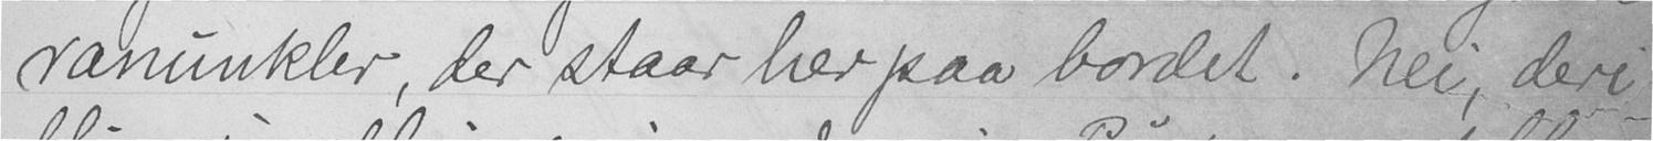ranunkler, der staar her paa bordet. Nei, deri
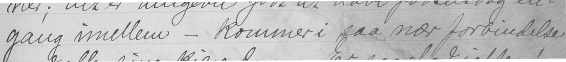gang imellem - kommer i saa nær forbindelse
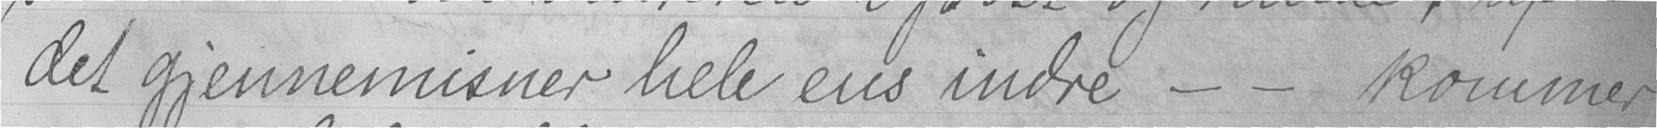det gjennemisner hele ens indre - - kommer
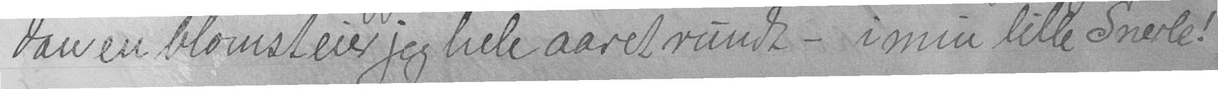dan en blomst eier jeg hele aaret rundt - i min lille Snerle!
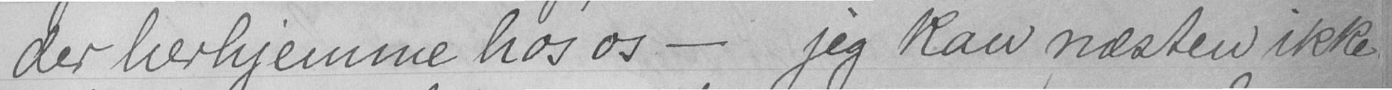der herhjemme hos os - jeg kan næsten ikke
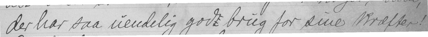der har saa uendelig godt brug for sine kræfter!
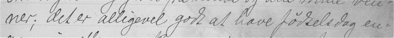ner; det er alligevel godt at have fødselsdag en-
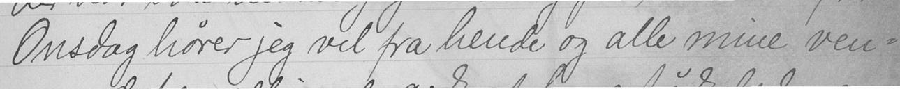Onsdag hører jeg vel fra hende og alle mine ven-
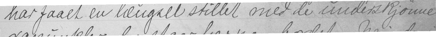har faaet en længsel stillet med de underskjønne
